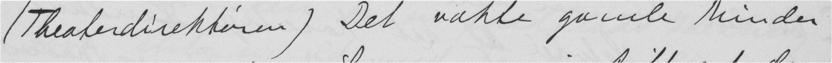(Theaterdirktøren) Det vækte gamle Minder
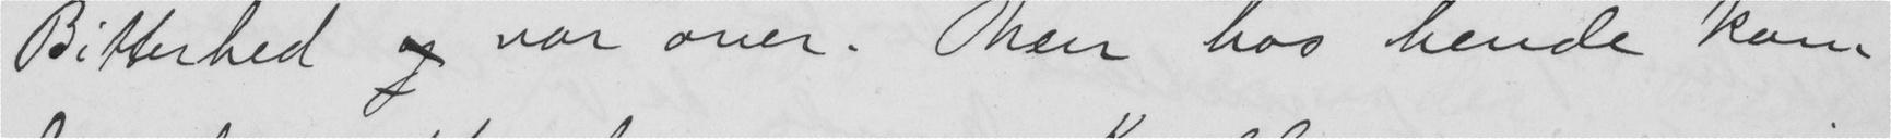Bitterhed  var over. Men hos hende kom
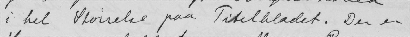i hel Størrelse paa Titelbladet. Der er
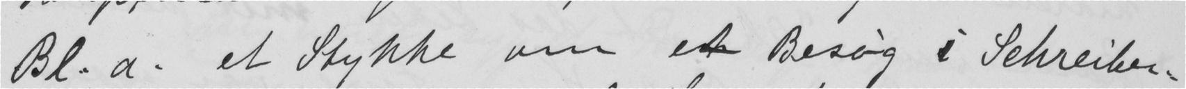Bl. a. et Stykke om et Besøg i Schreiber-
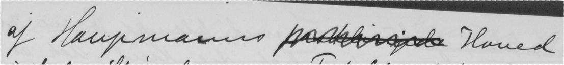af Haupmanns  Hoved
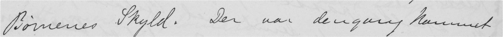Børnenes Skyld. Der var dengang kommet
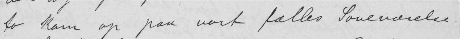to kom op paa vort fælles Soveværelse.
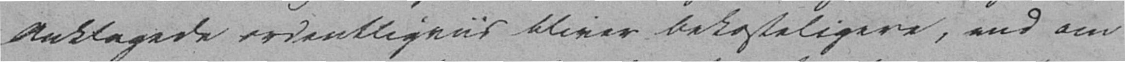Anklagede ordentligviis bliver bekosteligere, end om
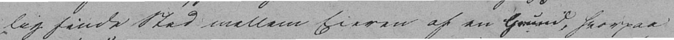lig finde Sted mellem Eieren af en Grund, hvorpaa
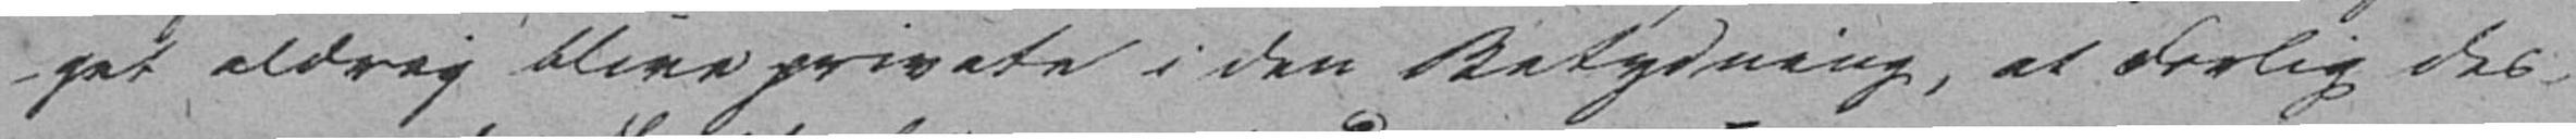get aldrig blive private i den Betydning, at Forlig des-
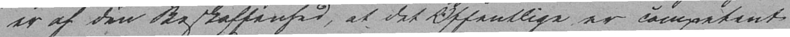er af den Beskaffenhed, at det Offentlige er competent
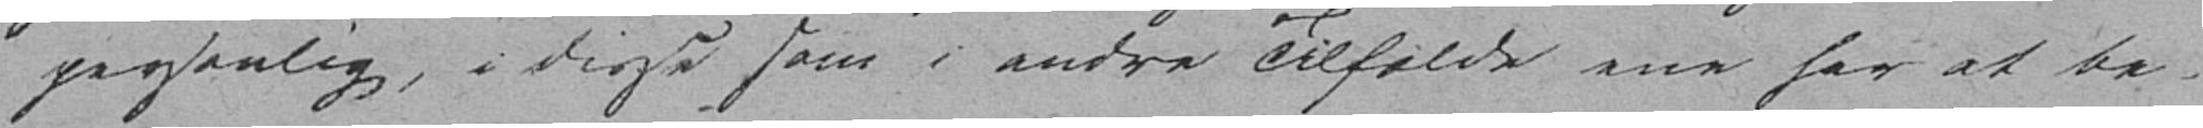personlig, i disse som i andre Tilfælde ene har at be-
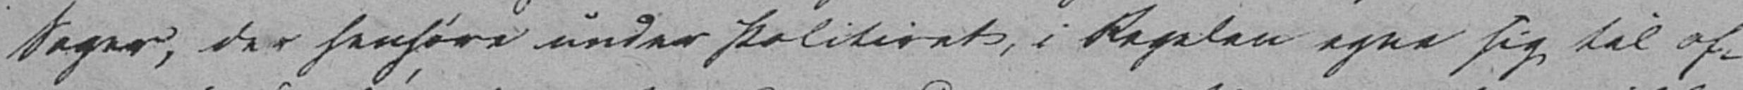Sager, der henhøre under Politiret, i Regelen egne sig til of-
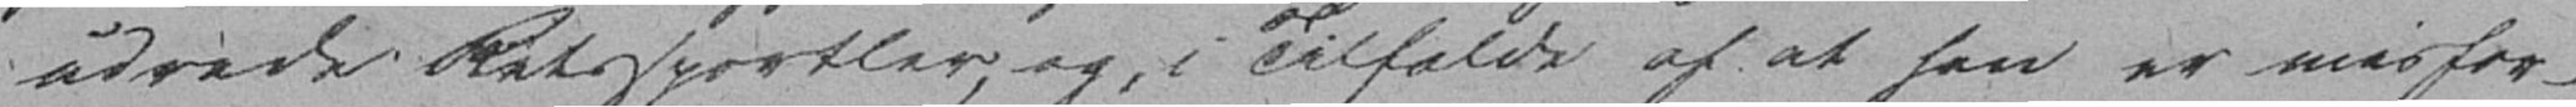udrede Retssportler, og i Tilfælde af at han er misfor-
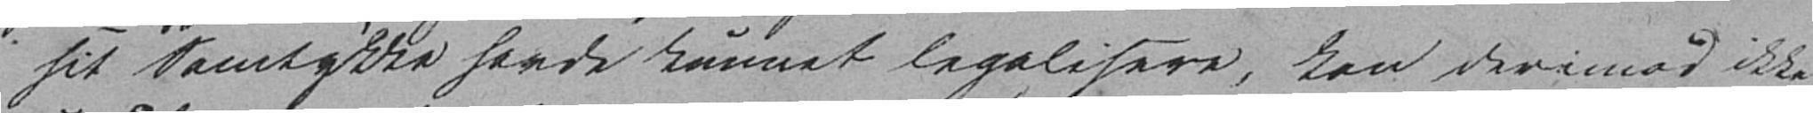sit samtykke havde kunnet legalisere, kan derimod ikke
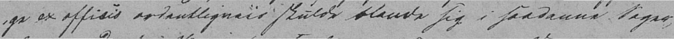ge ex officio ordentligvis skulde blande sig i saadanne Sager,
